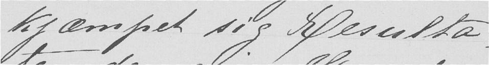kjæmpet sig Resulta-
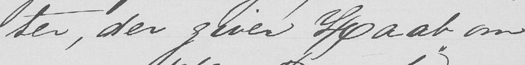ter, der giver Haab om
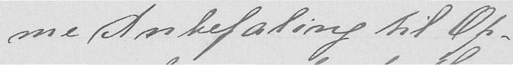me Anbefaling til Op-
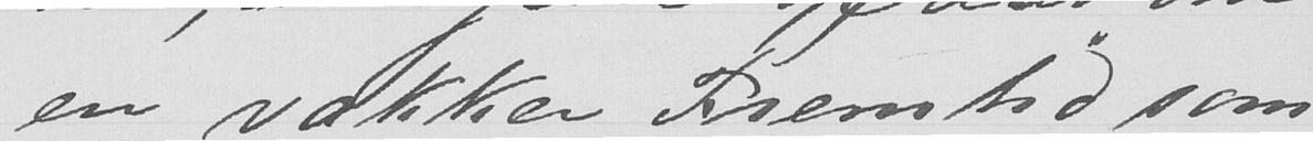en vakker Fremtid som
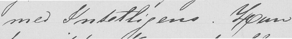med Intetligens. Hun
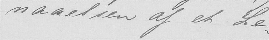naaelsen af et Le-
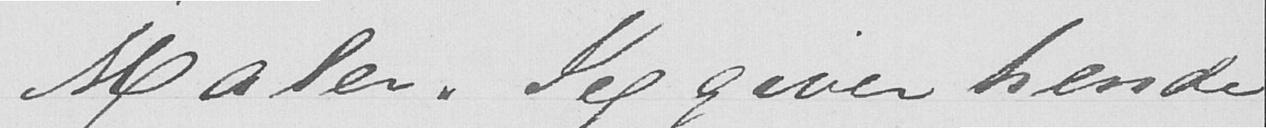Maler. Jeg giver hende
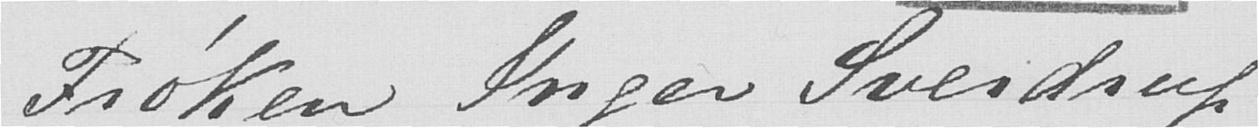Frøken. Inger Sverdrup
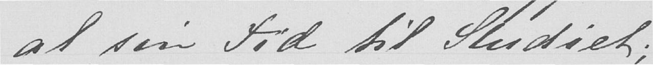af sin Tid til Studiet;
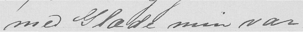med Glæde min var-
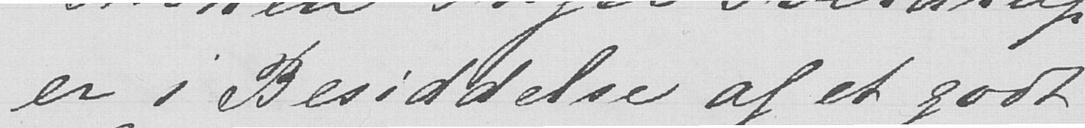er i Besiddelse af et godt
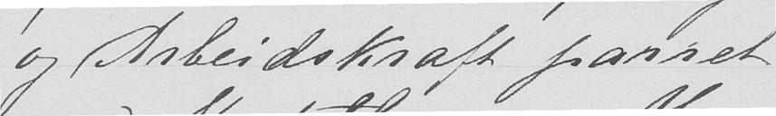og Arbeidskraft parret
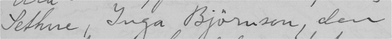Sethne, Inga Björnson, den
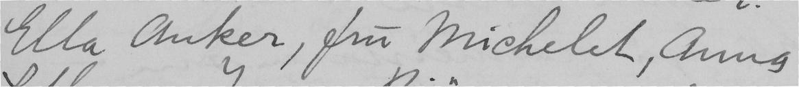Ella Anker, fru Michelet, Anna
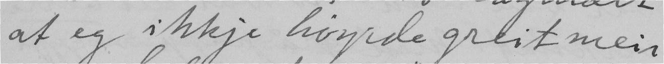at eg ikkje höyrde greit meir
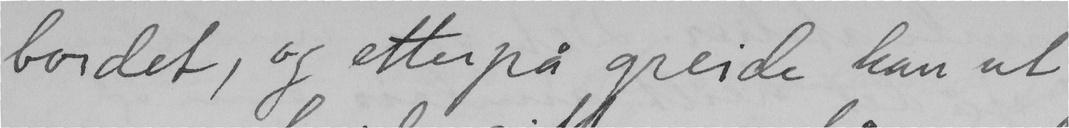bordet, og etterpå greide han at
